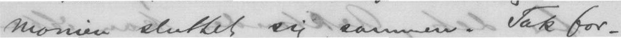monien sluttet sig sammen. Tak for
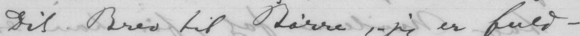Dit Brev til Børre, jeg er fuld-
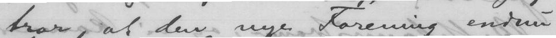tror, at den nye Forening endnu
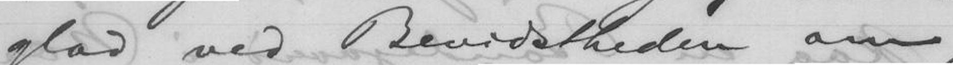glad ved Bevidstheden om,
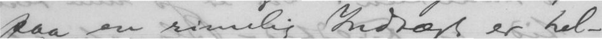paa en rimelig Indtækt er hel-
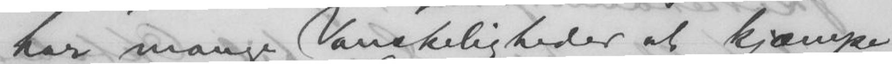har mange Vanskeligheder at kjæmpe
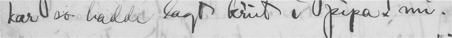kar so hadde lagt krut i pipa mi.
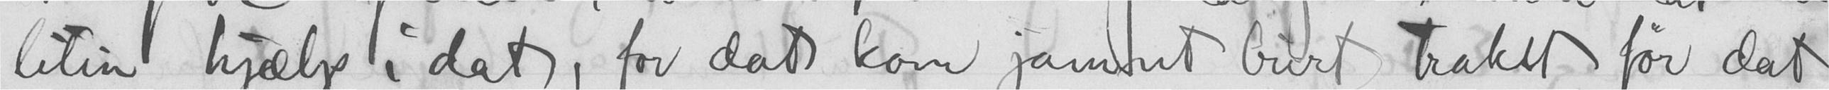litin hjælp i dat, for dat kom jamnt burt traket før dat
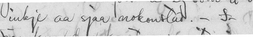inkje aa sjaa nokonstad. -
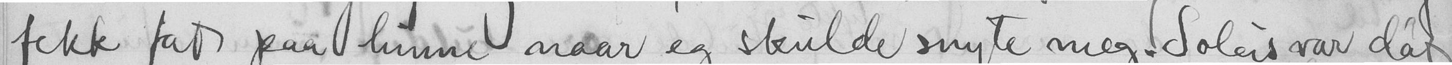fekk fat paa hinne naar eg skulde snyte meg. Soleis var dat
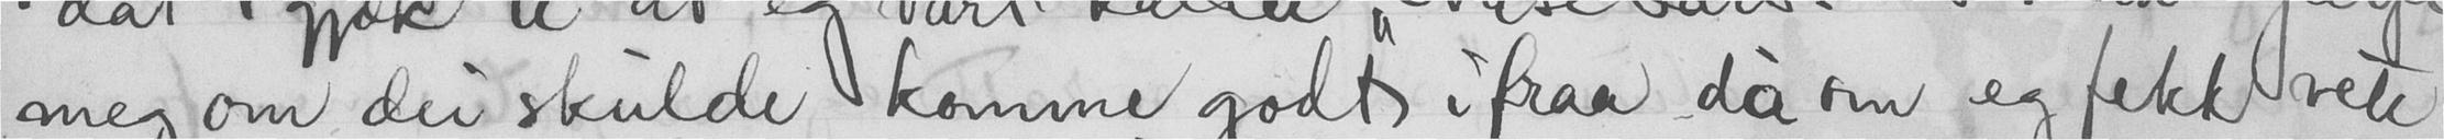meg om dei skulde komme godt ifraa da um eg fekk vete
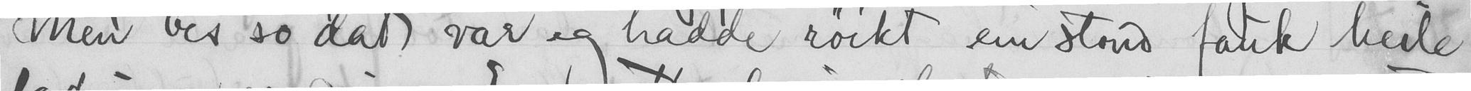Men bes so dat var eg hadde røikt ein stond fauk heile
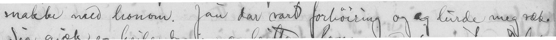snakke med honom. Jau dar vart forhøiring og eg lurde mig væke.
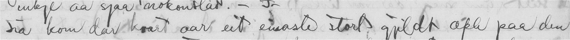Sia kom dar kvart aar eit einaste storts gjildt æple paa den
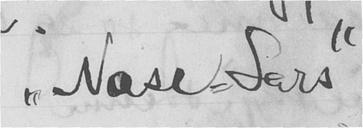"Nase-Lars"
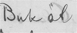Buk øl
